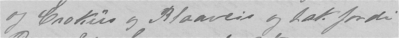og Crokus og Blaaveis og tak fordi
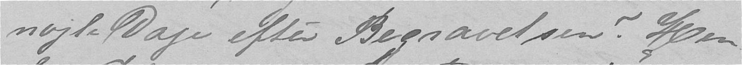nogle Dage efter Begravelsen? Hen-
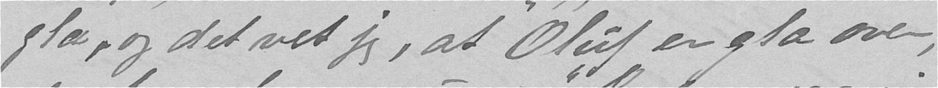gla, og det vet jeg, at Oluf er gla over,
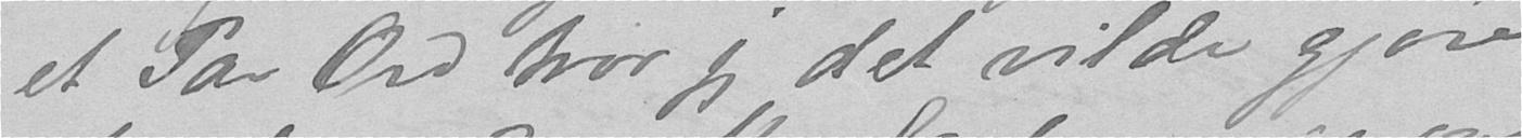et Par Ord tror jeg det vilde gjøre
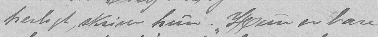herligt, skriver hun. Hun er bare
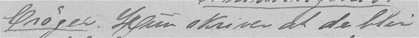Crøger. Hun skriver at de blir
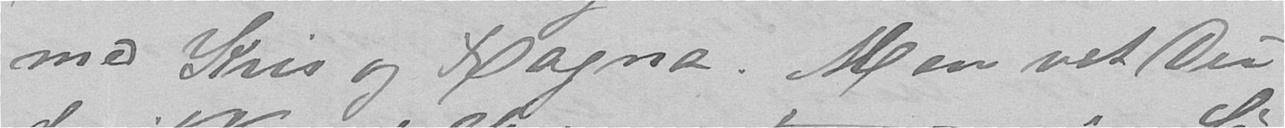med Kris og Ragna. Men vet Du
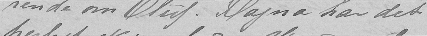rende om Oluf. Ragna har det
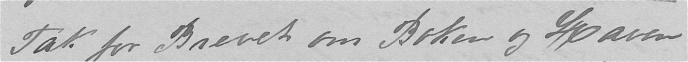Tak for Brevet om Boken og Haven-
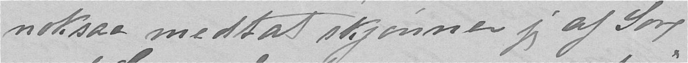noksaa medtat skjønner jeg af Sorg
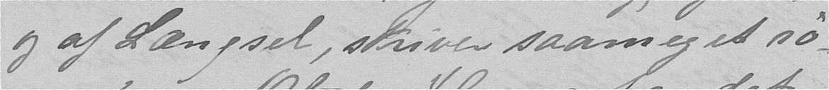og af Længsel, skriver saameget rø-
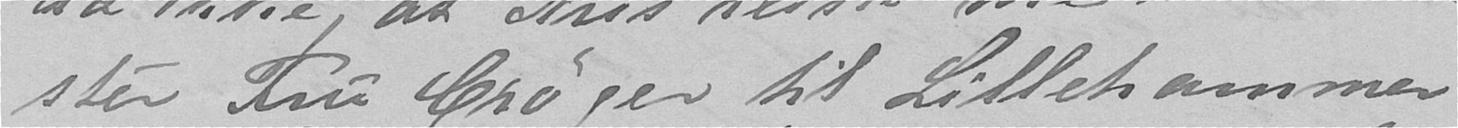ster Fru Crøger til Lillehammer
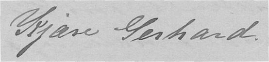Kjære Gerhard.
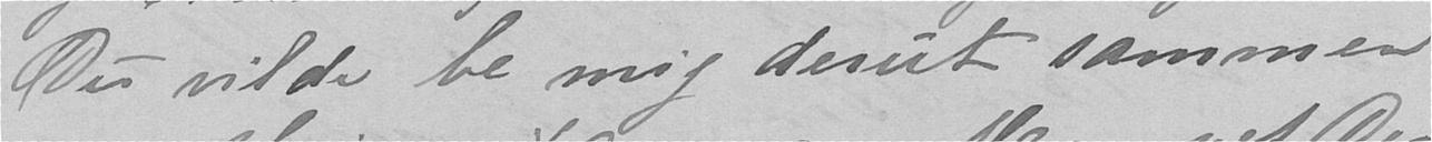Du vilde be mig derut sammen
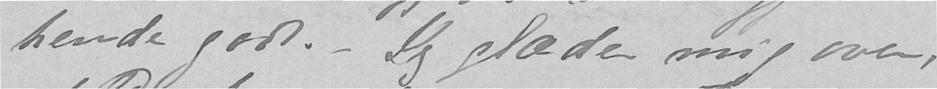hende godt. Jeg glæder mig over,
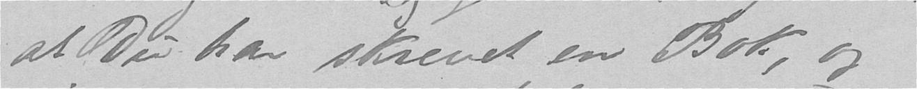at Du har skrevet en Bok, og
